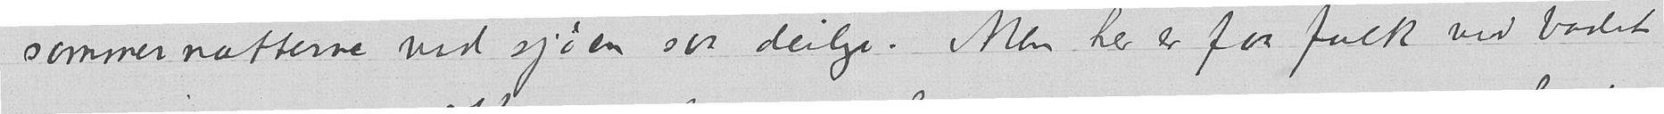sommernætterne ved sjøen saa deilige. Men her er faa folk ved badet
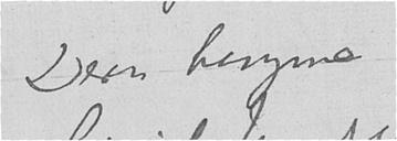Deres hengive
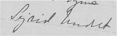Sigrid Undset
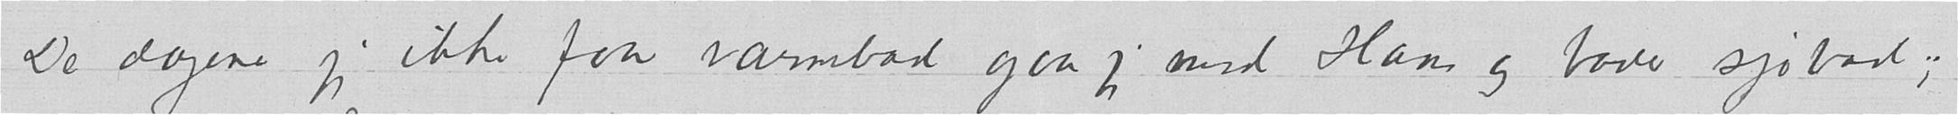De dagene jeg ikke faar varmbad gaar jeg med Hans og bader sjøbad;
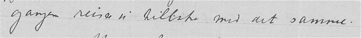gangen reiser vi tilbake med det samme.
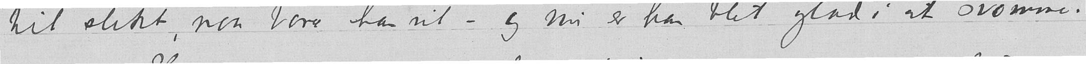til slikt, naar bare han vil – og nu er han blit glad i at svømme.
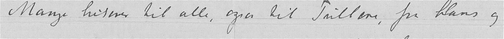Mange hilsener til alle, ogsaa til Tullene, fra Hans og
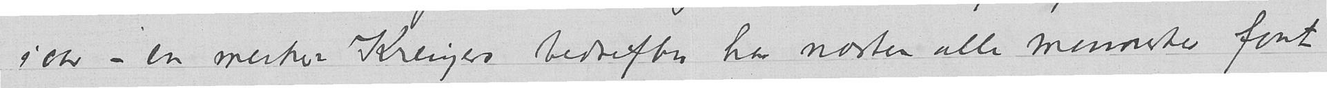iaar – en merker Kreugers bedrifter har næsten alle mennesker faat
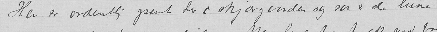Her er ordentlig pent her i skjærgaarden og saa er de lune
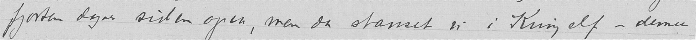fjorten dager siden ogsaa, men da stanset vi i Kungelf – denne
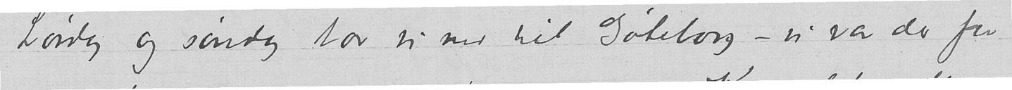Lørdag og søndag tar vi ned til Gøteborg – vi var der for
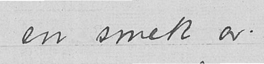en smek av.
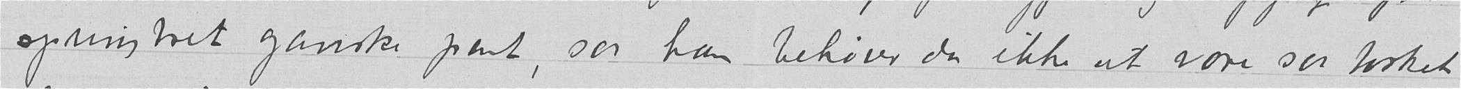springbret ganske pent, saa han behøver da ikke at være saa tosket
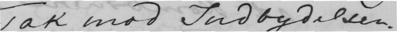Tak mod Indbydelsen.
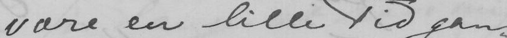være en lille Tid gan
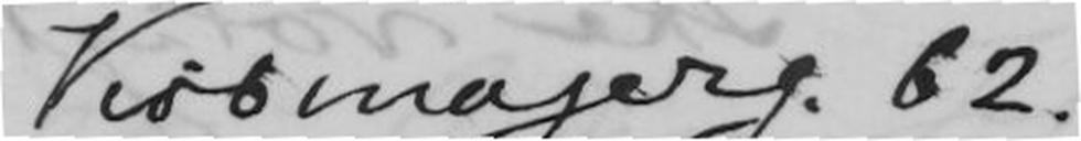Viosmagerg. 62
